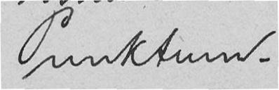Punktum.
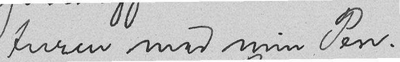turen med min Pen.
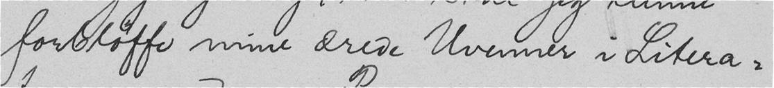forbløffe mine ærede Uvenner i Litera-
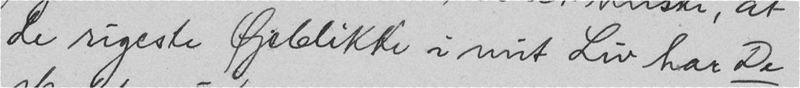de rigeste Øjeblikke i mit Liv har De
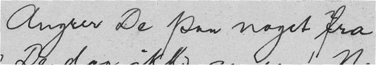Angrer De paa noget fra
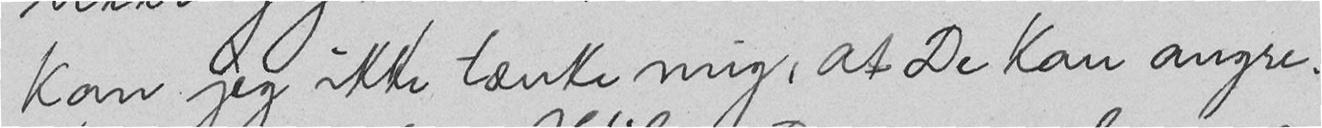kan jeg ikke tænke mig, at De kan angre.
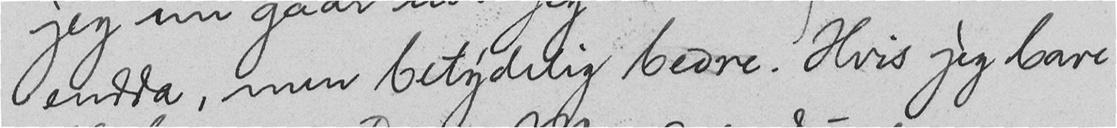endda, men betydelig bedre. Hvis jeg bare
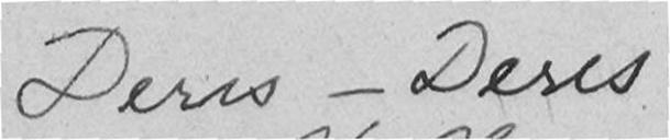Deres - Deres
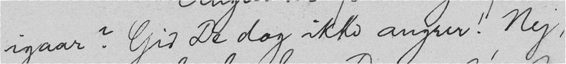igaar? Gid De dog ikke angrer! Nej,
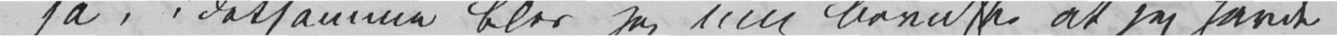ja, i detsamme blev jeg mig bevidst at jeg havde
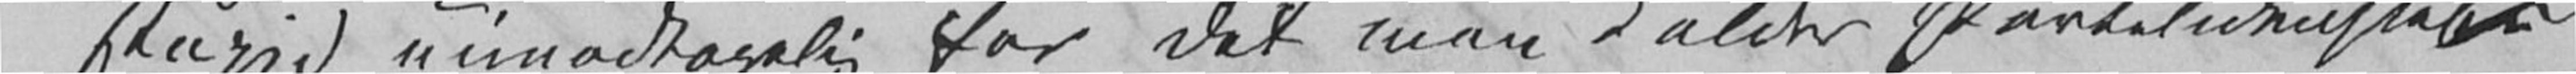stupid uimodtagelig for det man kalder Partilidenskab
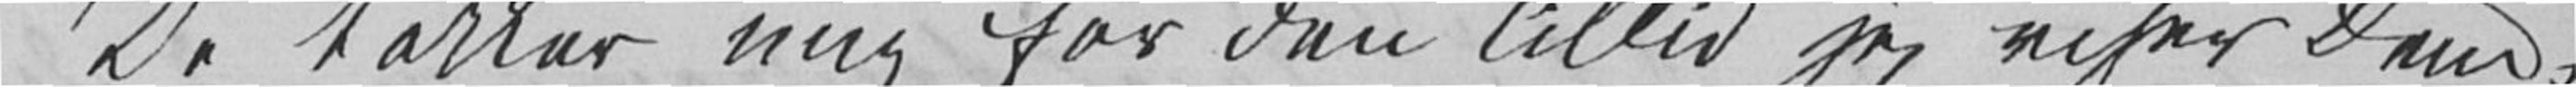De takker mig for den Tillid jeg viser Dem,
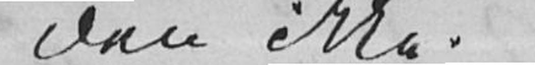den ikke.
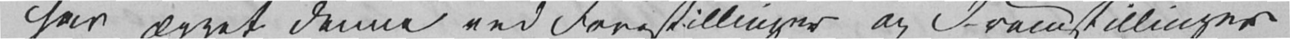har ægget denne ved Forestillinger og Fremstillinger
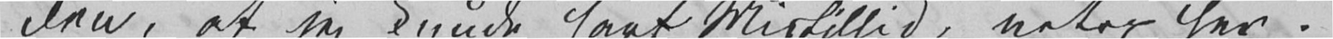den, at jeg kunde havt Mistillid, netop her.
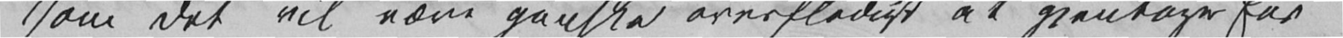som det vil være ganske overflødigt at gjentage for
